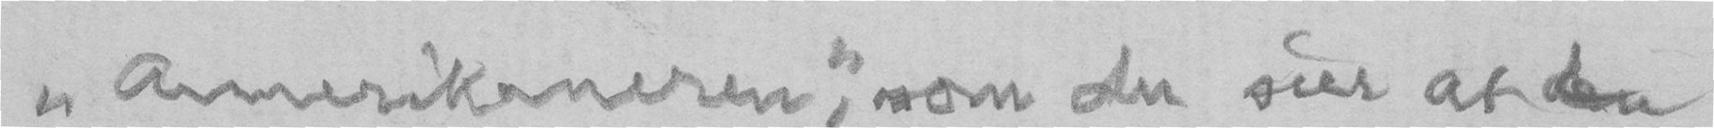"Amerikaneren", som du sier at du
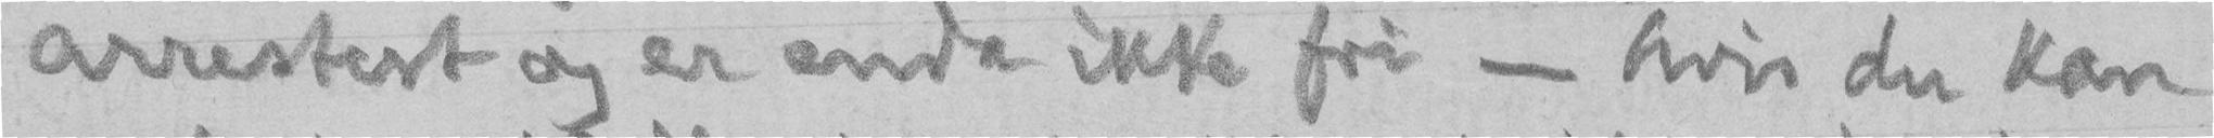arrestert og er enda ikke fri - hvis du kan
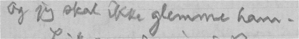og jeg skal ikke glemme ham.
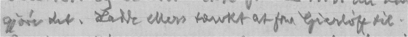gjøre det. Hadde ellers tænkt at faa Gierløff til.
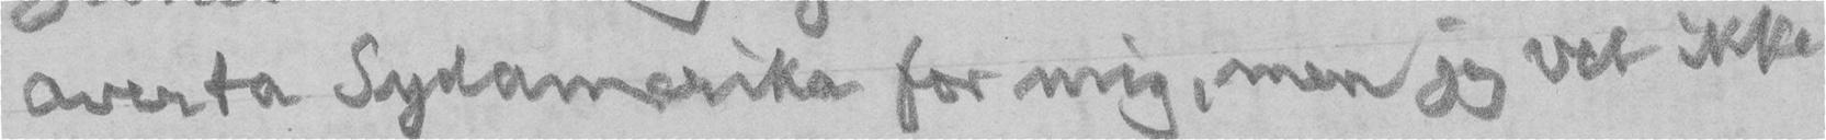overta Sydamerika for mig, men jeg vet ikke
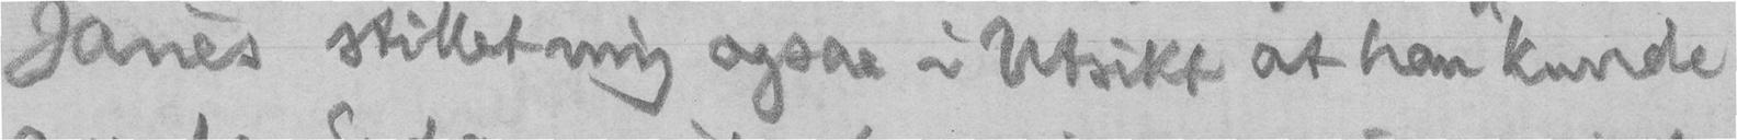Janès stillet mig ogsaa i Utsikt at han kunde
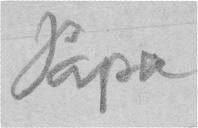Papa
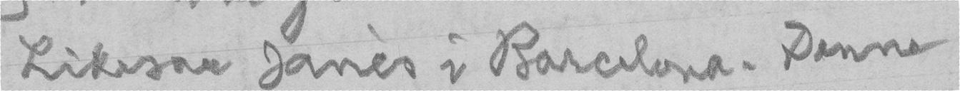Likesaa Janès i Barcelona. Denne
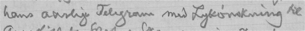hans aarlige Telegram med Lykønskning til
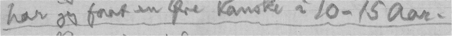har jeg faat en Øre kanske i 10-15 Aar.
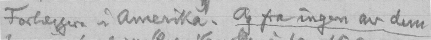Forlæggere i Amerika. Og fra ingen av dem
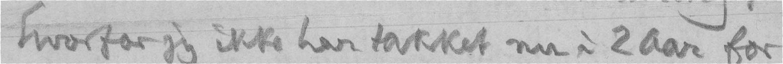hvorfor jeg ikke har takket nu i 2 Aar for
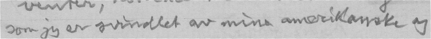som jeg er svindlet av mine amerikanske og
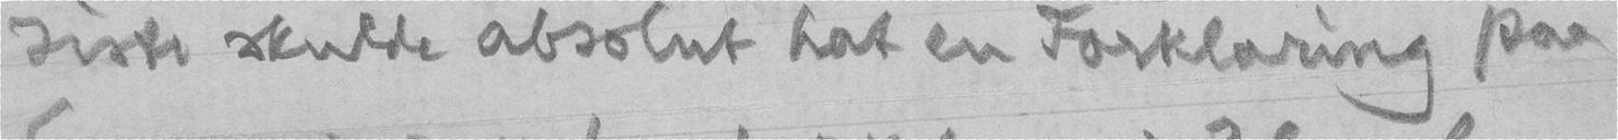siste skulde absolut hat en Forklaring paa
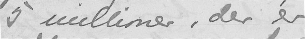5 millioner, der er
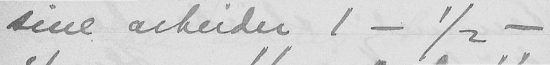sine arbeider 1 - ½ -
